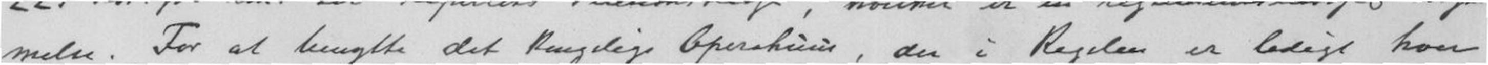melse. For at benytte det Opera, der i Regelen er ledigt hver
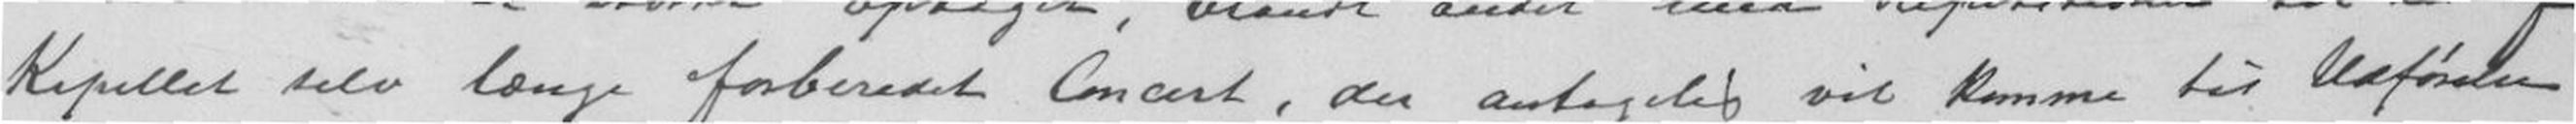Kapellet selv længe forberedet Concert, der antagelig vil komme til Udførelse
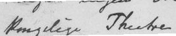Kongelige Theatre.
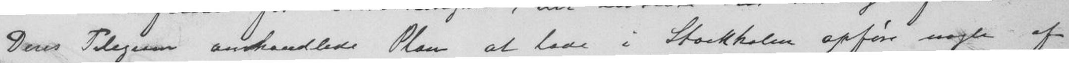Deres Telegram om Plan at lade i Stockholm opføre nogle af
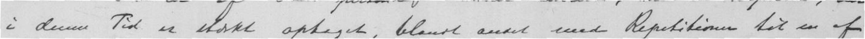i denne Tid er optaget, blandt andel med Repetioner til en af
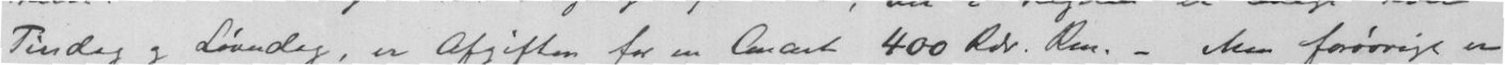Tirsdag og Lørdag, er Afgiften for en Concert 400 Rdr. Ren.- Men forøvrige er
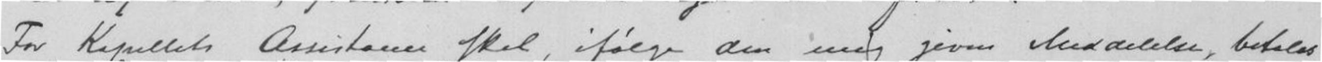For Kapellets Assistanse skal, ifølge den mig given Meddelelse, befales
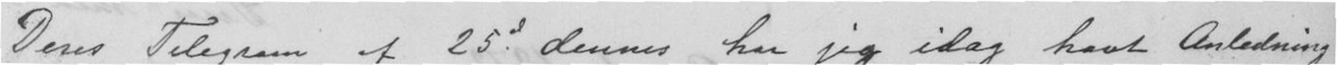Deres Telegram af 25de dennes har jeg idag havt Anledning
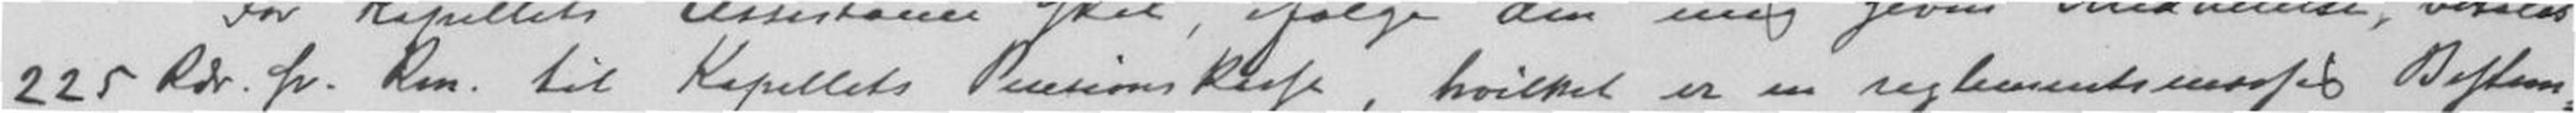225 Rdr. P. Kun til Kapellets , hvilket er en reg Bestem-
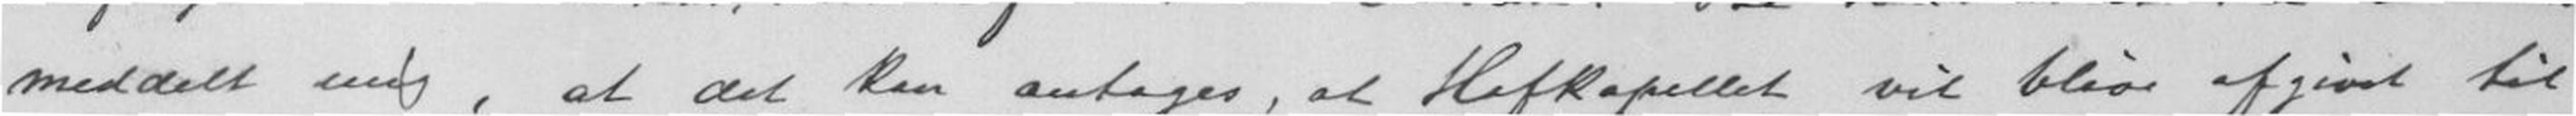meddelt mig, at det kan antages, at Hofkapellet vil blive afgivet til
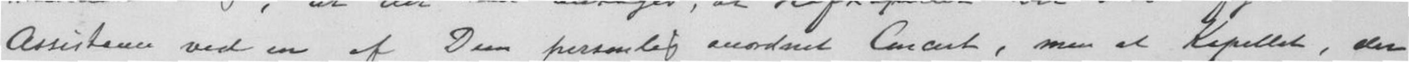ved en af Dem personlig anordnet Concert, men at Kapellet, der
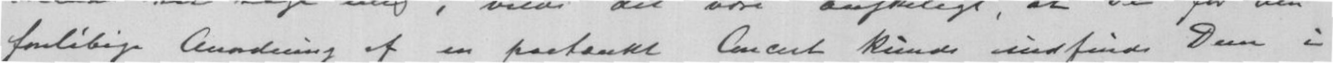Anmodning af en paatænkt Concert kunde indfinde Dem i
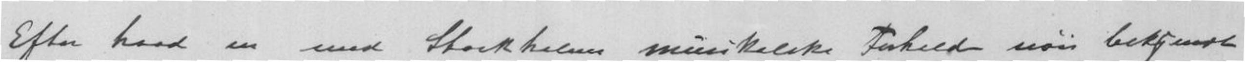Efter hvad en med Stockholm musikalske Forhold nøie bekjendt
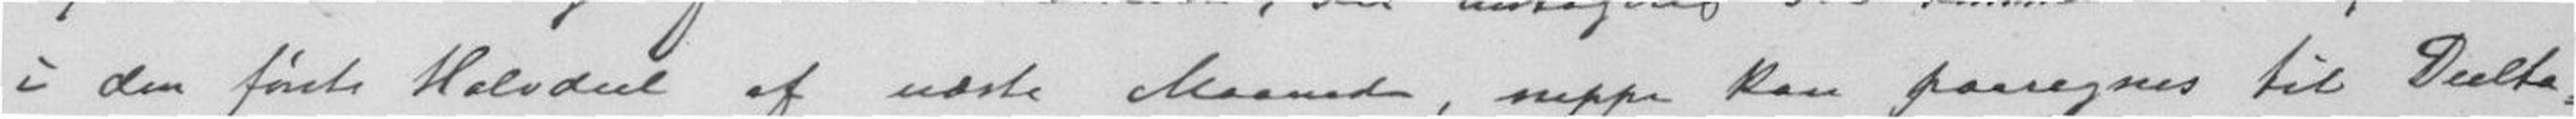i den første Halvdeel af næste Maanede, neppe kan paaregnes til Deelta-
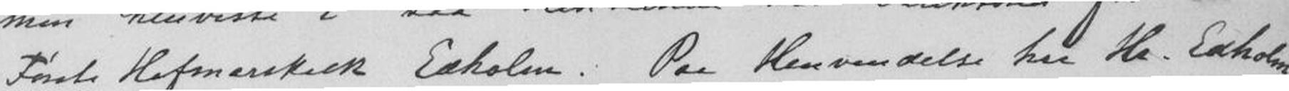Første Hofmarskeck E.holm. Paa Henvendelse har H. E.holm
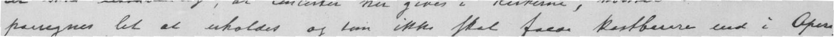paaregnes let at uholdes og som ikke skal falde kortberera end i Opera-
